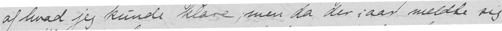af hvad jeg kunde klare; men da der i aar meldte sig
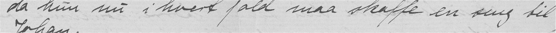da hun nu i hvert fald maa skaffe en seng til
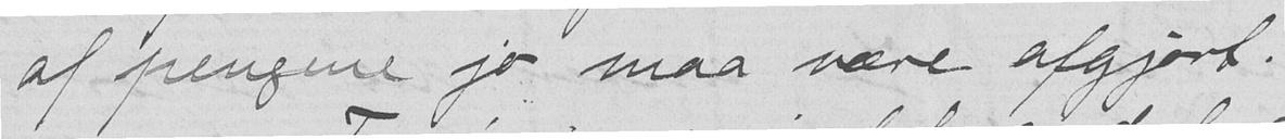af pengene jo maa være afgjort.
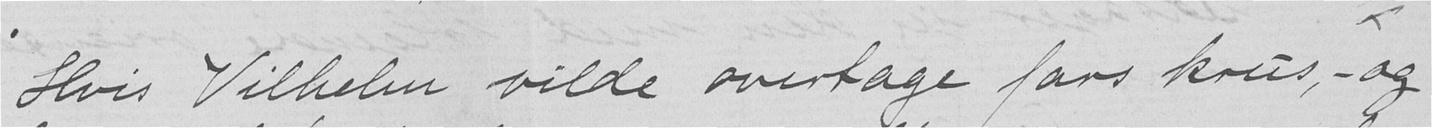Hvis Vilhelm vilde overtage fars krus, - og
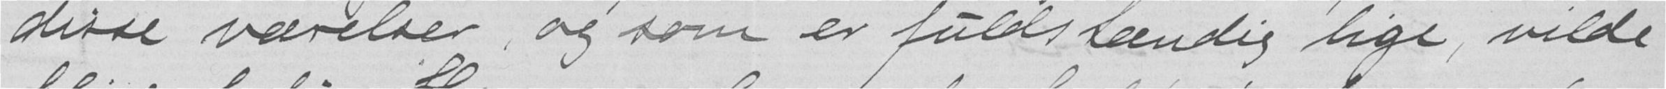disse værelser, og som er fuldstændig lige, vilde
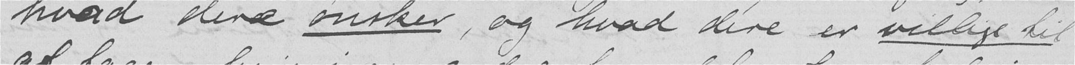hvad dere ønsker, og hvad dere er villige til
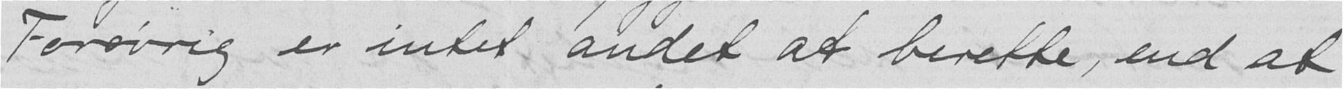Forøvrig er intet andet at berette, end at
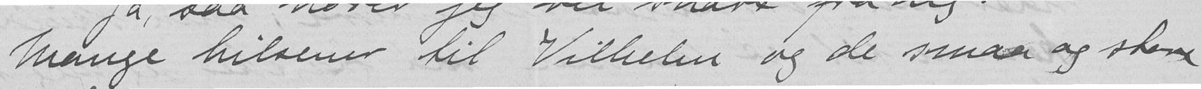Mange hilsener til Vilhelm og de smaa og store
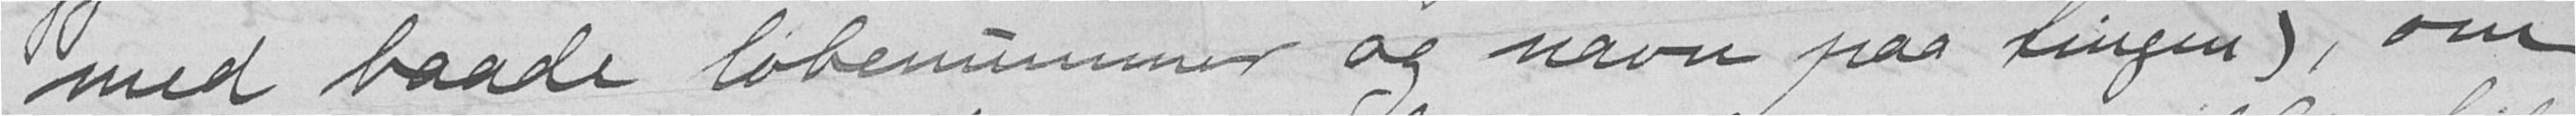med baade løbenummer og navn paa tingen), om
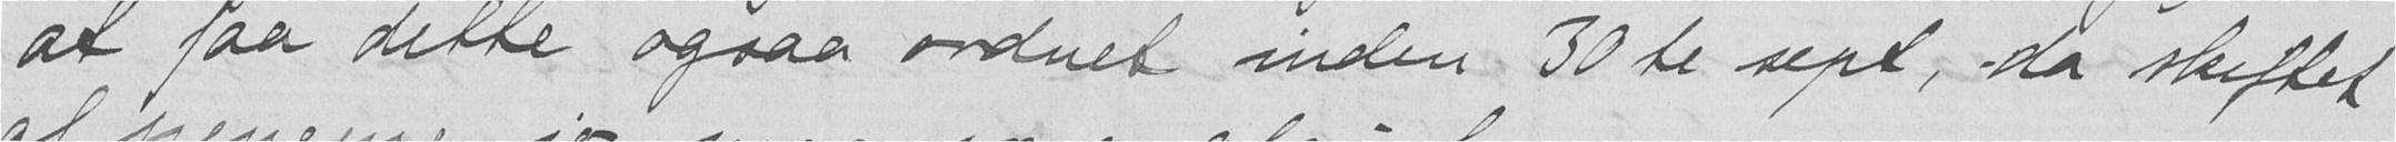at faa dette ogsaa ordnet inden 30te sept, da skiftet
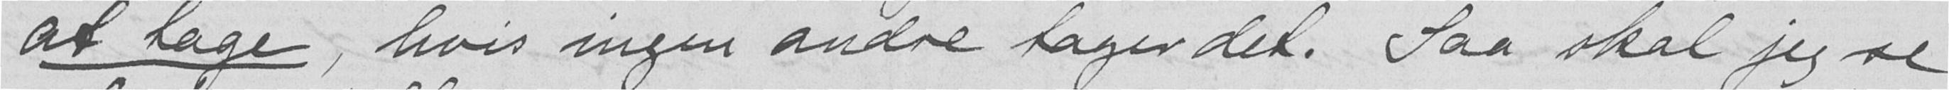at tage, hvis ingen andre tager det. Saa skal jeg se
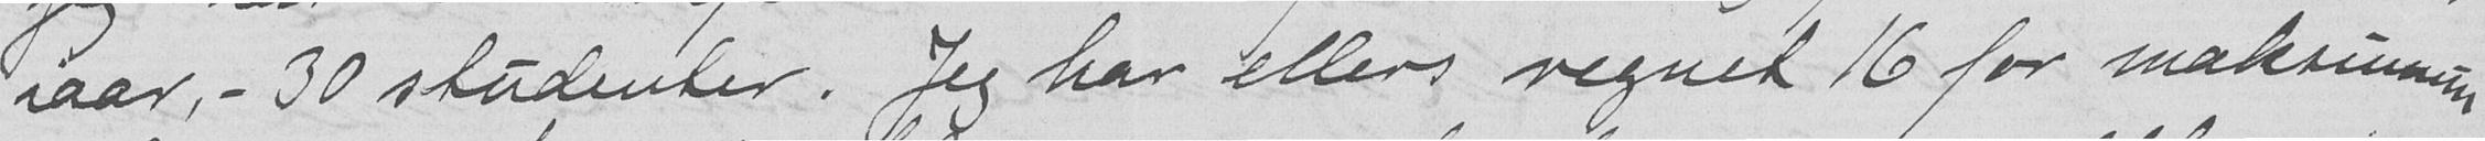iaar, - 30 studenter. Jeg har ellers regnet 16 for maksimum
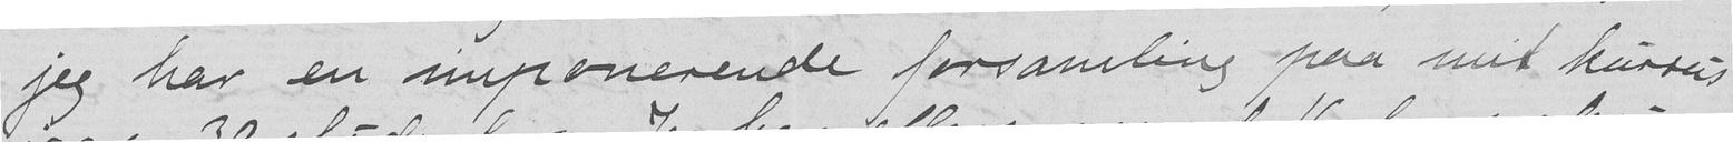jeg har en imponerende forsamling paa mit kursus
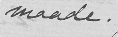maade.
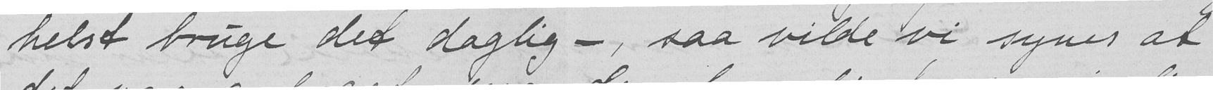helst bruge det daglig -, saa vilde vi synes at
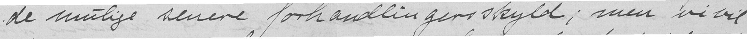de mulige senere forhandlingers skyld; men vi vil
